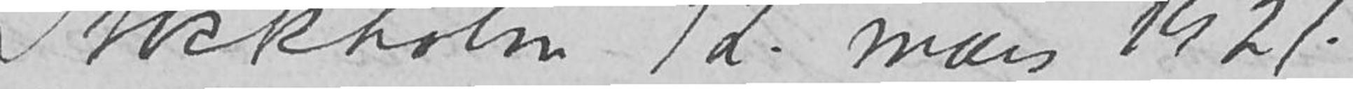Stockholm 12. mars 1921.
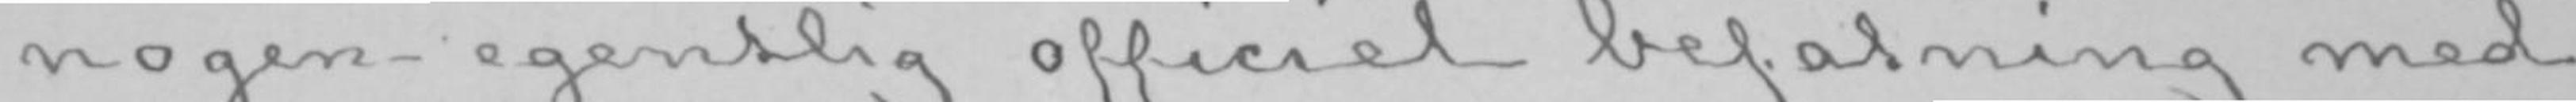nogen egentlig officiel befatning med
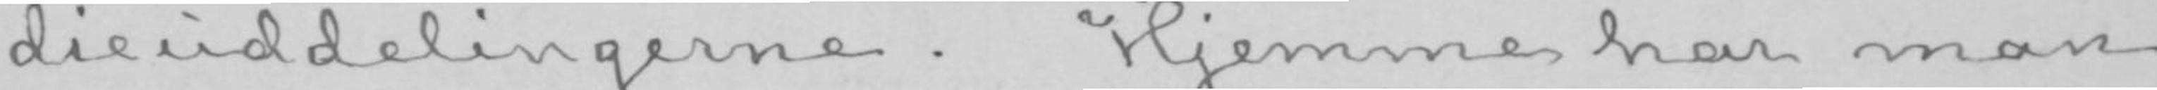dieuddelingerne. Hjemme har man
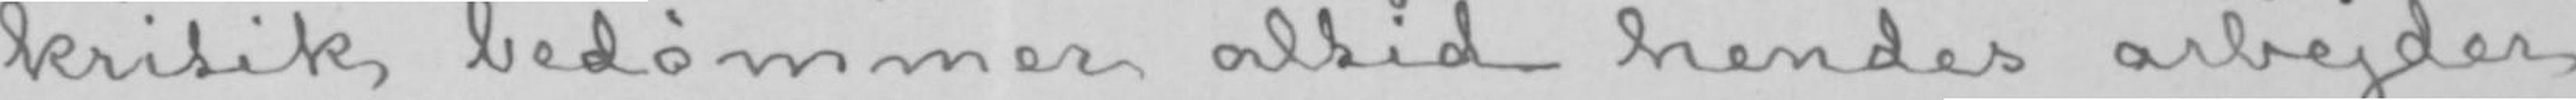kritik bedømmer altid hendes arbejder
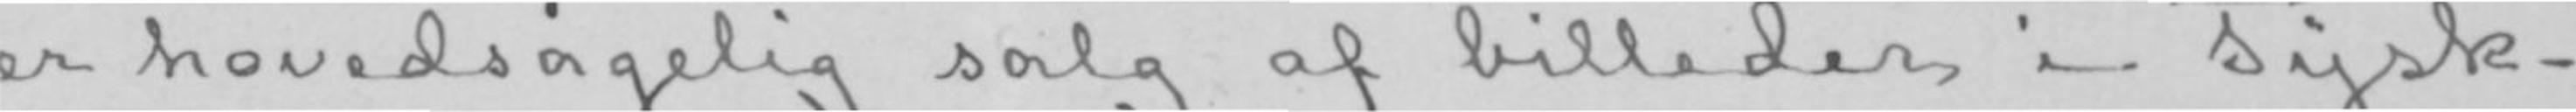er hovedsagelig salg af billeder i Tysk-
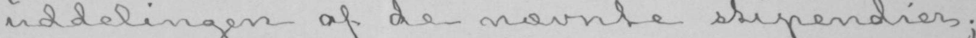uddelingen af de nævnte stipendier;
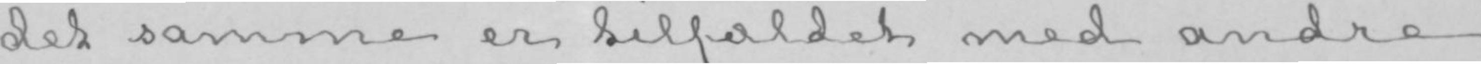det samme er tilfældet med andre
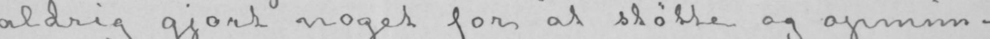aldrig gjort noget for at støtte og opmun-
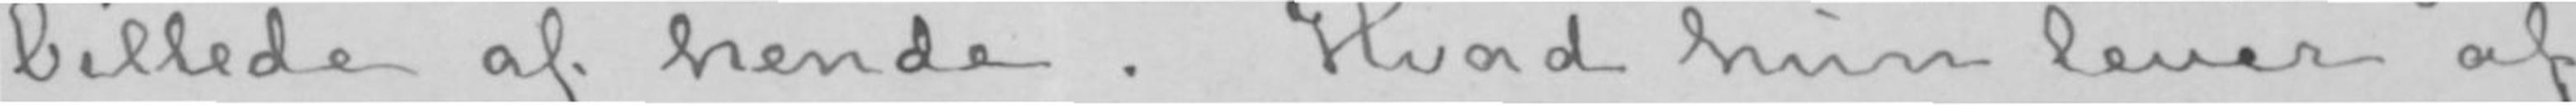billede af hende. Hvad hun lever af
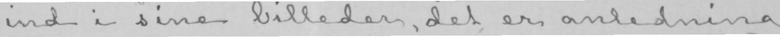ind i sine billeder, det er anledning
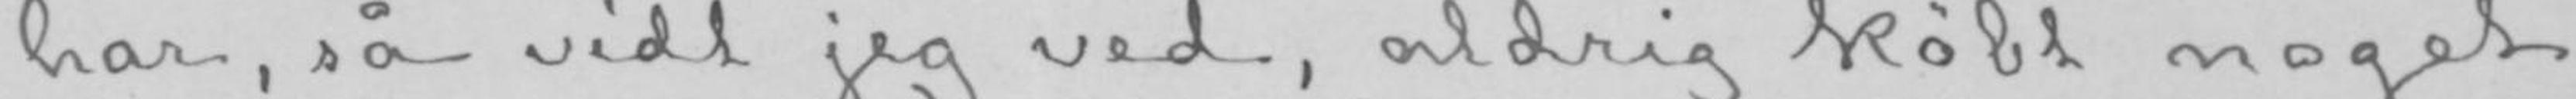har, så vidt jeg ved, aldrig købt noget
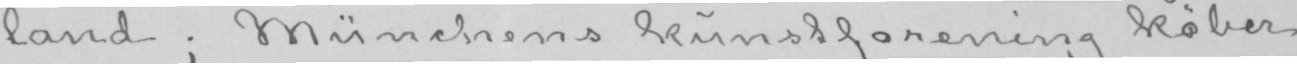land; Münchens kunstforening køber
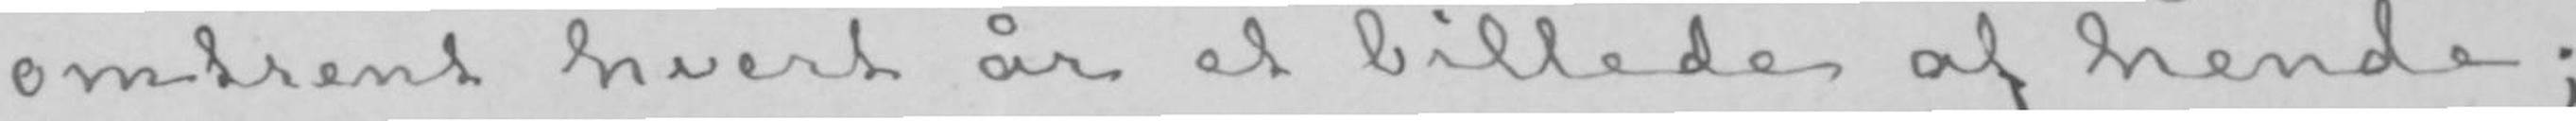omtrent hvert år et billede af hende;
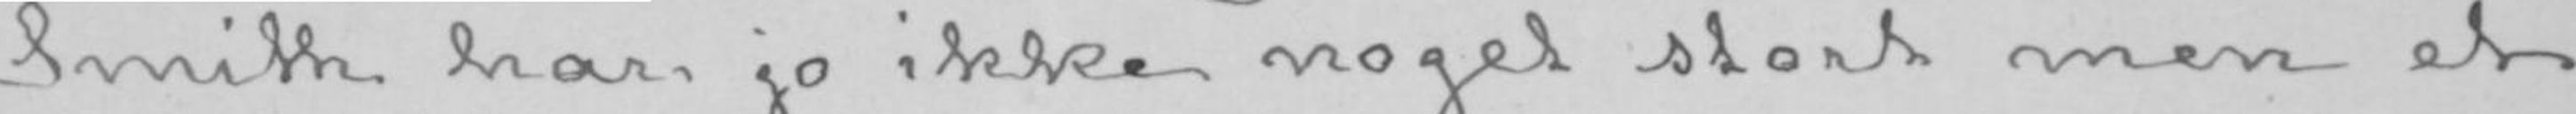Smith har jo ikke noget stort men et
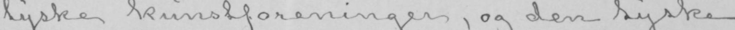tyske kunstforeninger, og den tyske
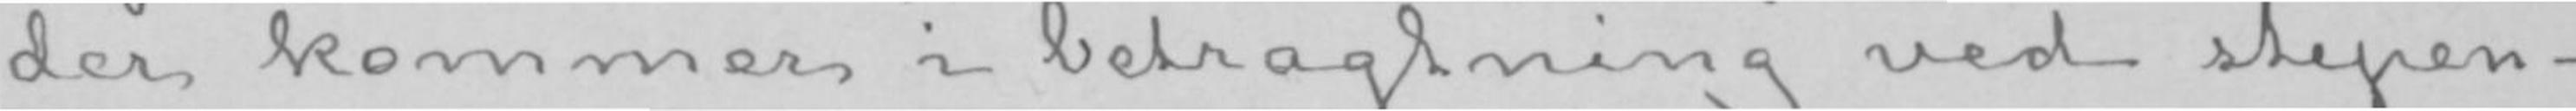der kommer i betragtning ved stipen-
